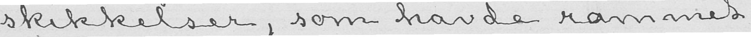skikkelser, som havde rammet
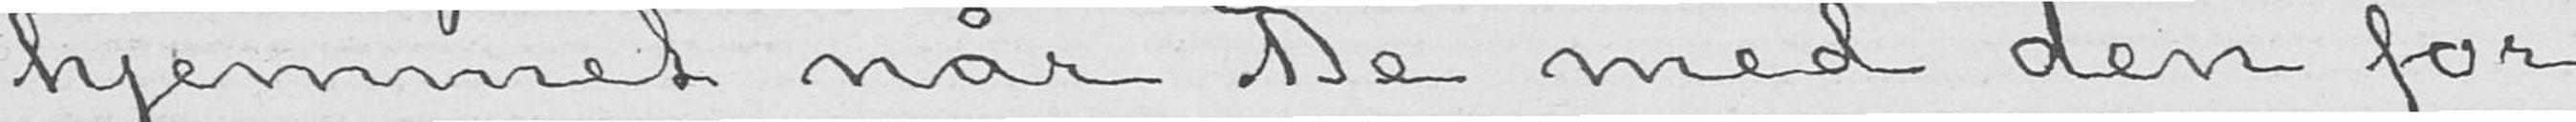hjemmet når De med den for
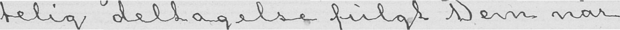telig deltagelse fulgt Dem når
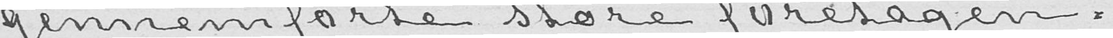gennemførte store foretagen-
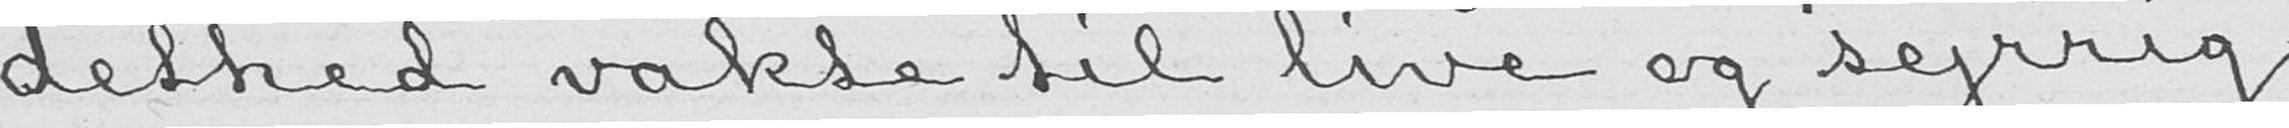dethed vakte til live og sejrrig
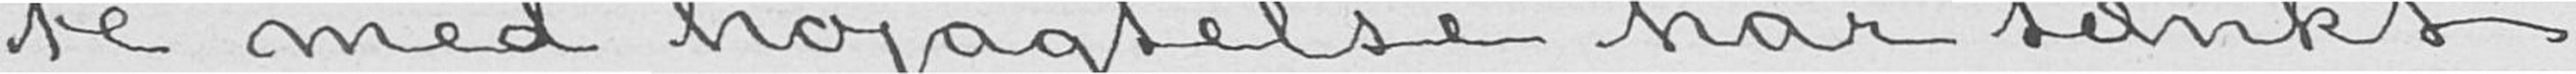te med højagtelse har tænkt
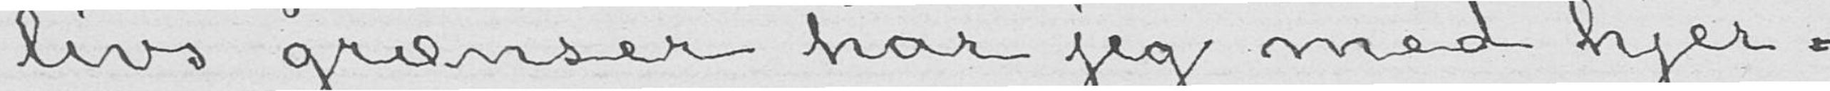livs grænser har jeg med hjer-
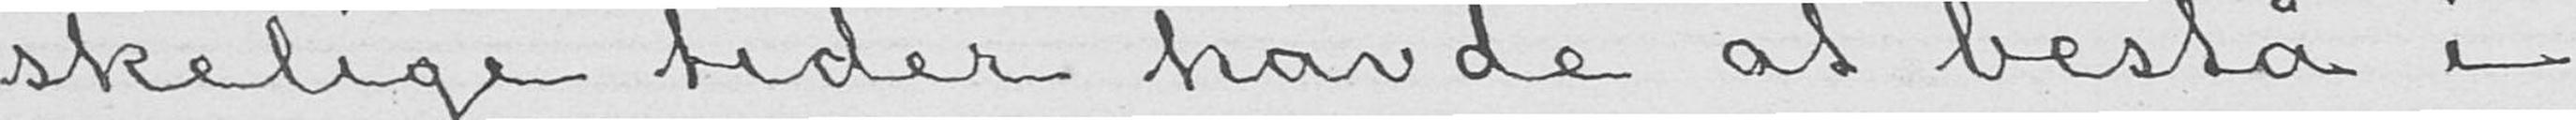skelige tider havde at bestå i
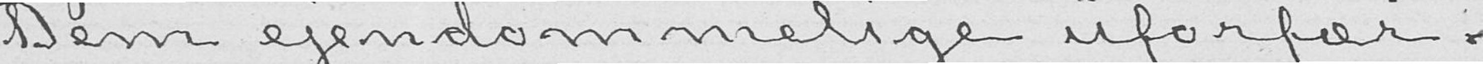Dem ejendommelige uforfær-
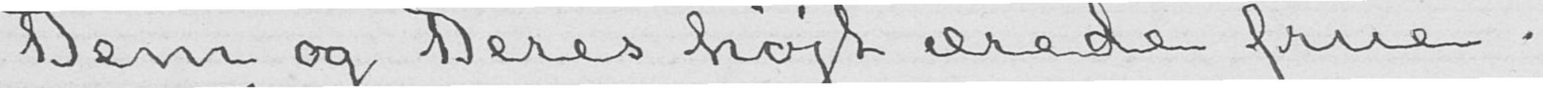Dem og Deres højt ærede frue.
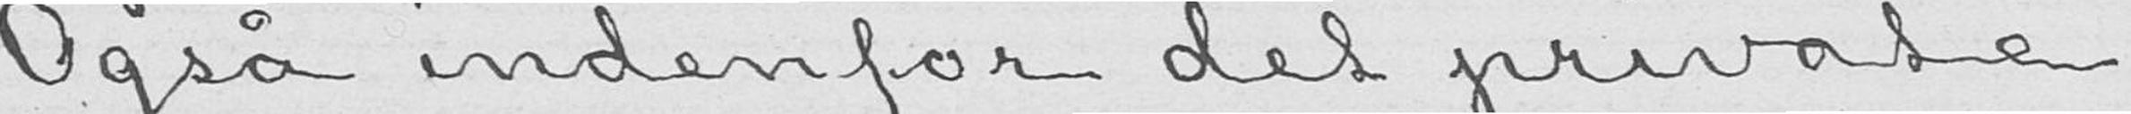Også indenfor det private
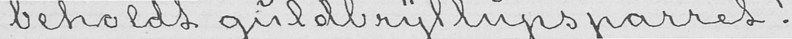beholdt guldbryllupsparret!
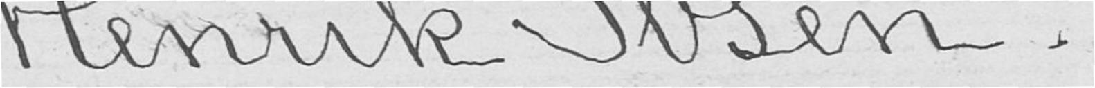Henrik Ibsen.
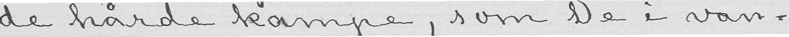de hårde kampe, som De i van-
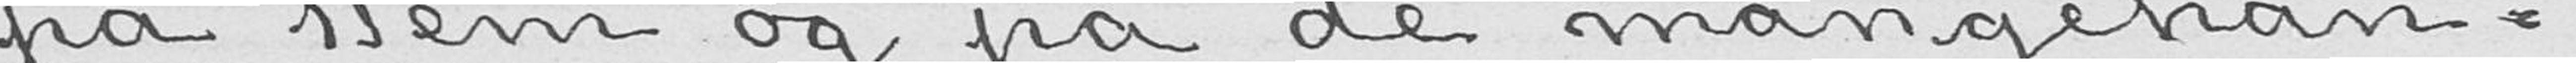på Dem og på de mangehån-
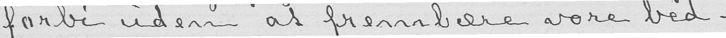forbi uden at frembære vore bed-
